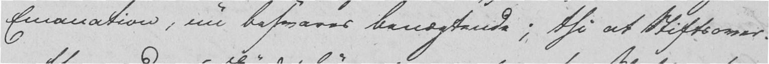Emanation, nu besvares benægtende; thi at Stiftsover-
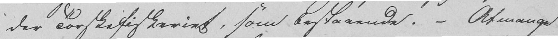der Torskefiskeriet, som bestaaende. - At mange
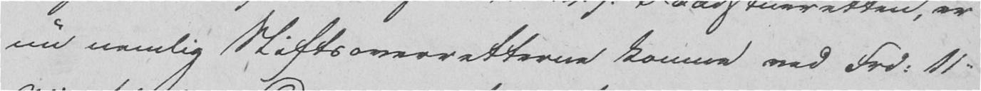nu nemlig Stiftsoverretterne komne ved Frd. 11.
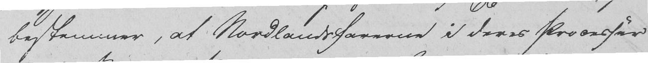bestemmer, at Nordlandsfarerne i deres Processer
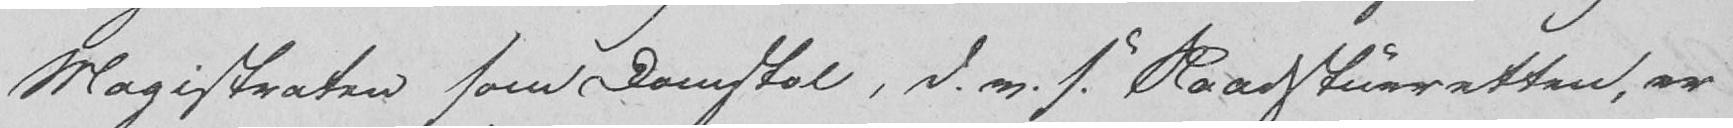Magistraten som Domstol, d. v. s. Raadstueretten, er
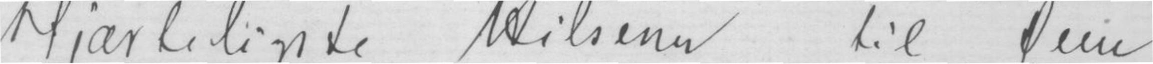Hjærteligste Hilsener til Dem
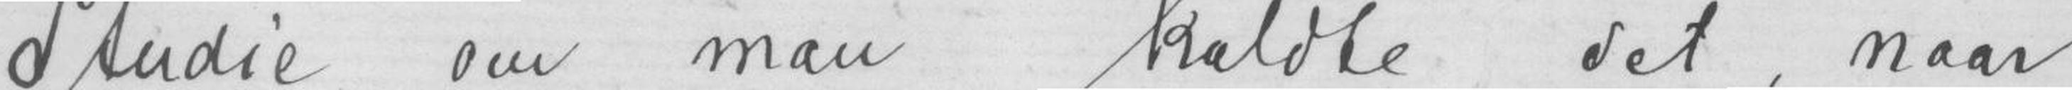Studie om man kaldte det, naar
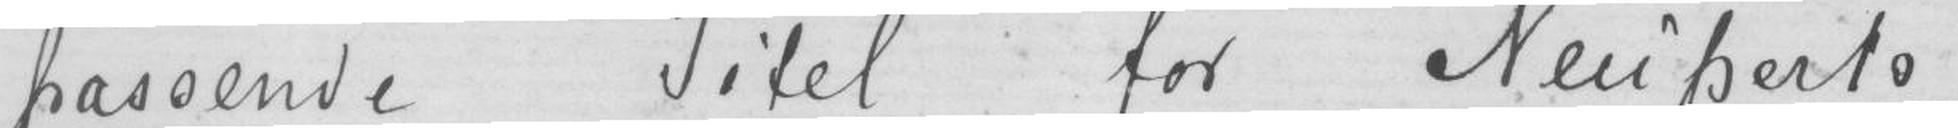passende Titel for Neuperts
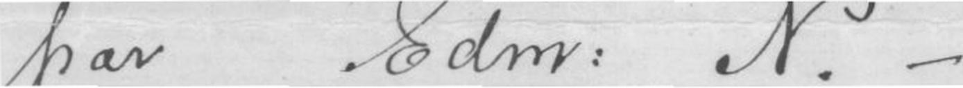par Edm: N.-
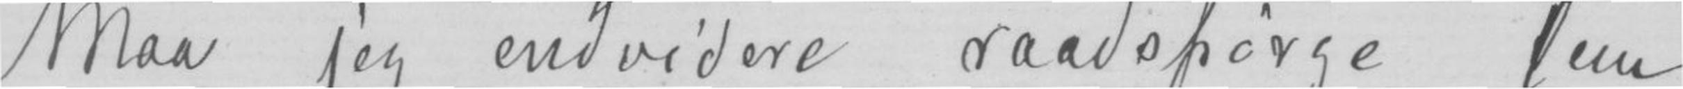Maa jeg endvidere raadspørge Dem
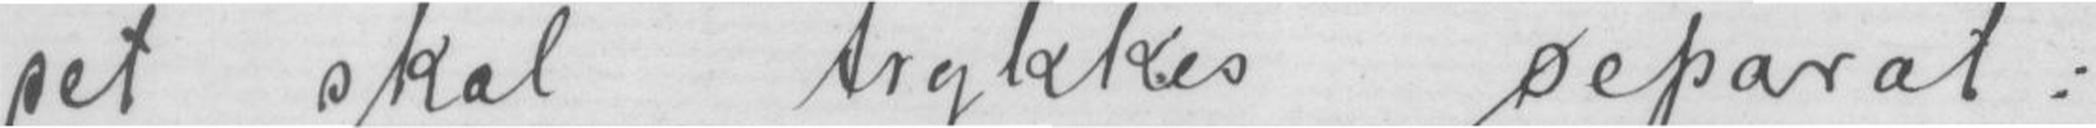det skal trykkes separert:
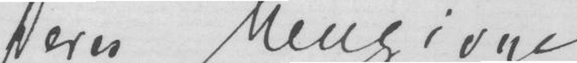Deres hengivne
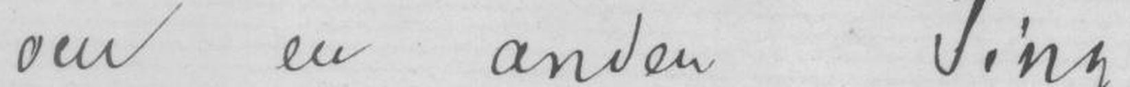om en anden Ting:
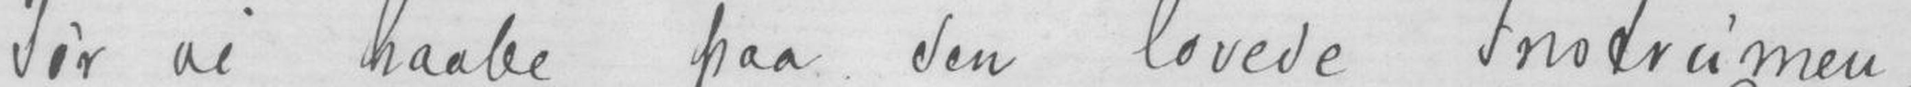Tør vi haabe paa den lovende Instrumen-
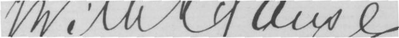Wilhelm Hansen
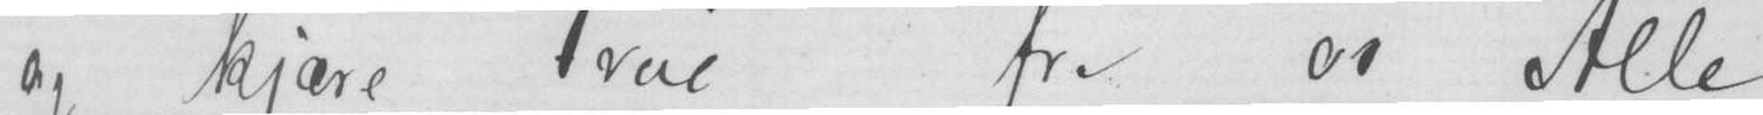og kjære frue fra os Alle
